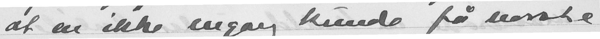at en ikke engang kunde få norske
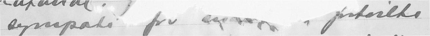sympati for sga, og fortvilte
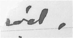søat,
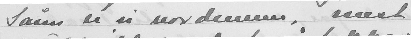Sånn er vi nordmenn, mest
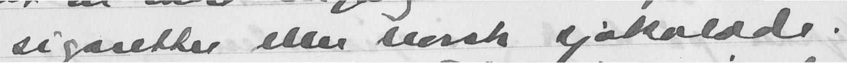sigaretter eller svensk sjokolade.
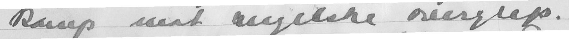kamp mot engelske overgrep.
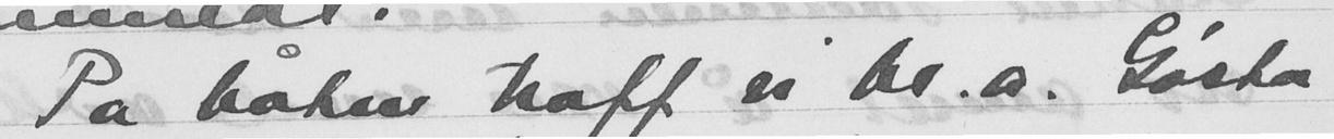På båten traff vi bl. a. Gøsta
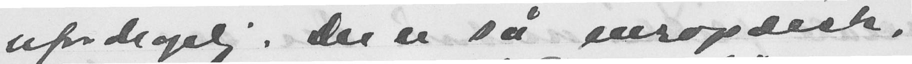ufordragelig. Der er så europeisk,
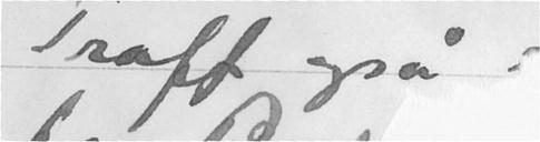Traff også
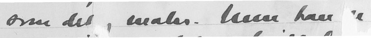som det, maler. Men han
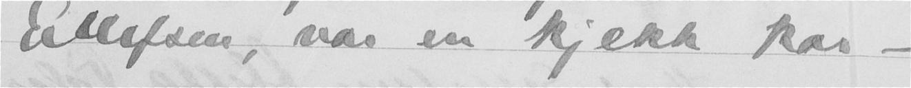Ellefsen, var en kjekk kar -
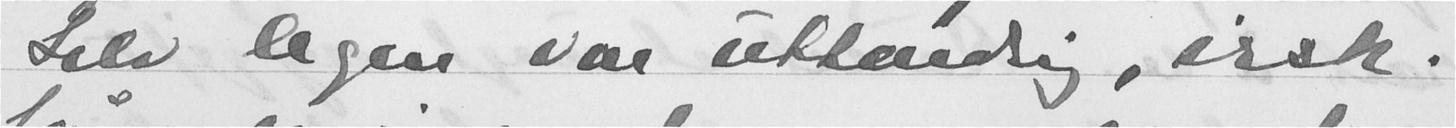Selv legen var utlending, irsk.
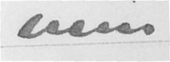sien
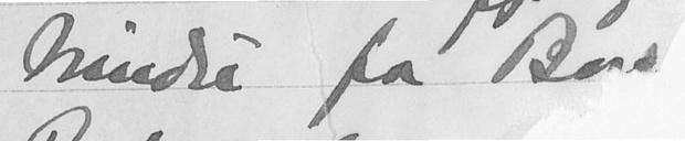Nandre fra Børs
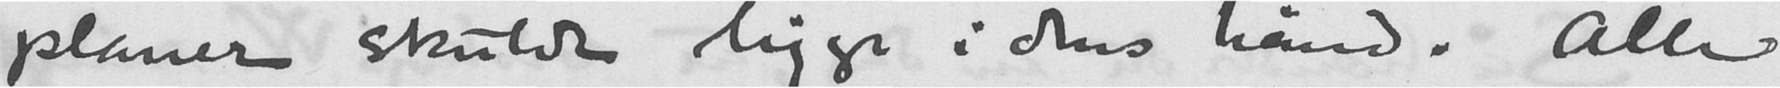planer skulde ligge i dens hånd. Alle
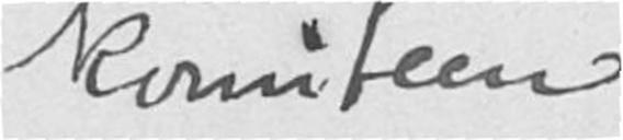komiteen
